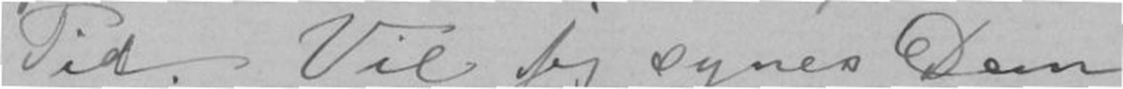Tid. Vil jeg synes Dem
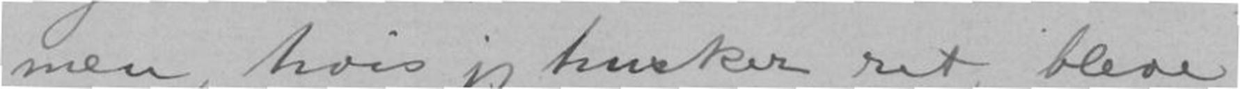men, hvis jeg husker ret, bleve
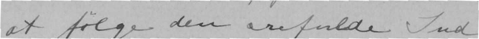at følge den ærefulde Ind-
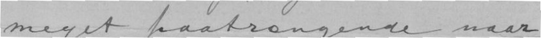meget paatrængende naar
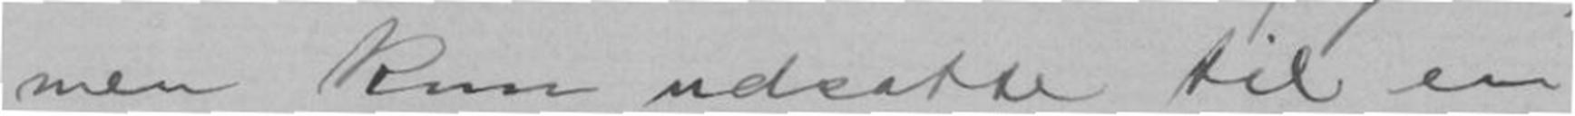men kun udsatte til en
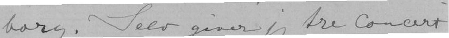borg. Selv giver jeg tre Concert-
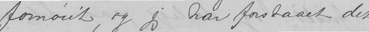fornøiet, og har forstaaet det
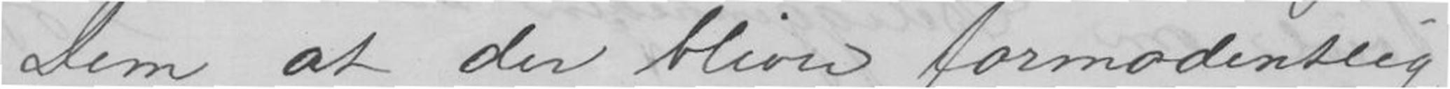Dem, at der bliver formodentlig
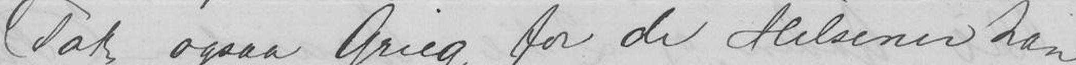Tak ogsaa Grieg for de Helsinger han
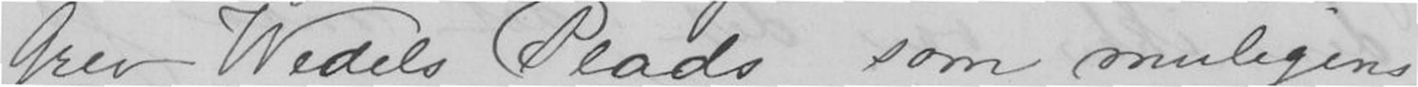Grev Wedels Plads, som muligens
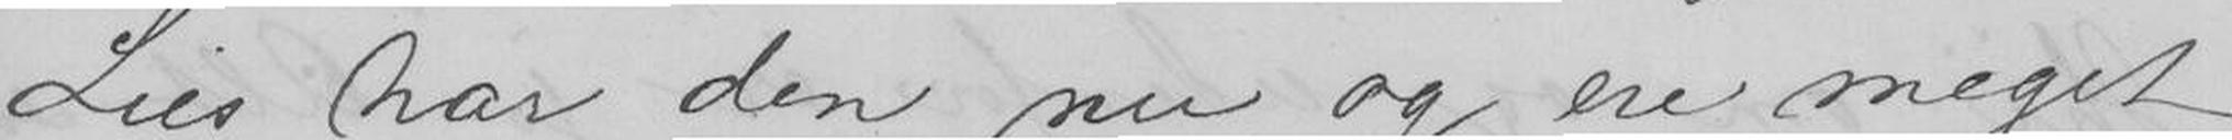Lies har den nu, og ere meget
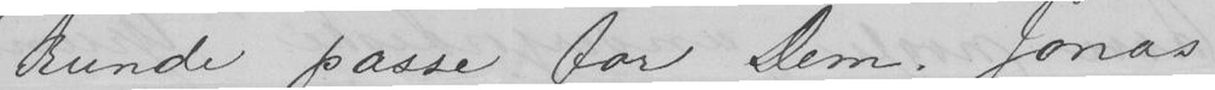kunde passe for Dem. Jonas
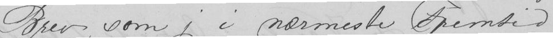Brev, som jeg i nærmeste Fremtid
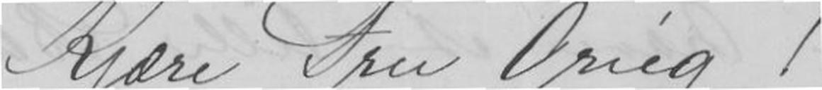Kjære Fru Grieg!
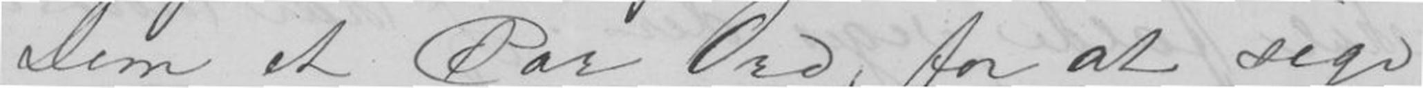Dem et Par Ord, for at sige
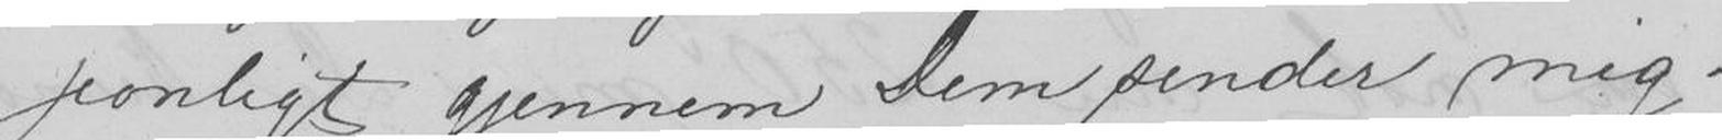personligt gjennem Dem sender mig.
